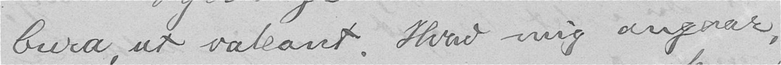bura, ut valeant. Hvad mig angaar,
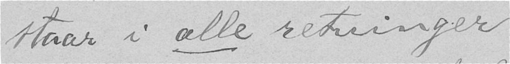staar i alle retninger.
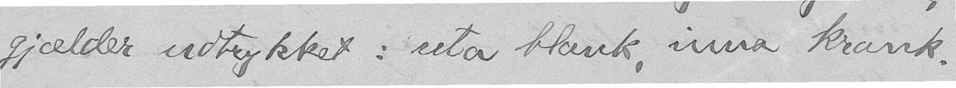gjælder udtrykket: uta blank, inna krank.
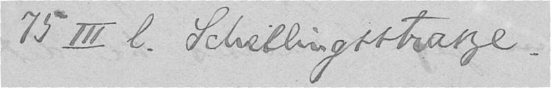75 III l. Schillingsstrasse.
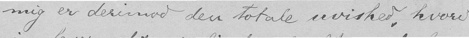mig er derimod den totale uvished, hvori
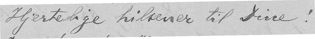Hjertelige hilsener til Dine!
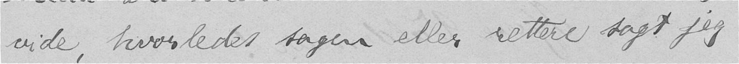vide, hvorledes sagen eller rettere sagt jeg
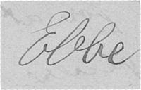Ebbe.
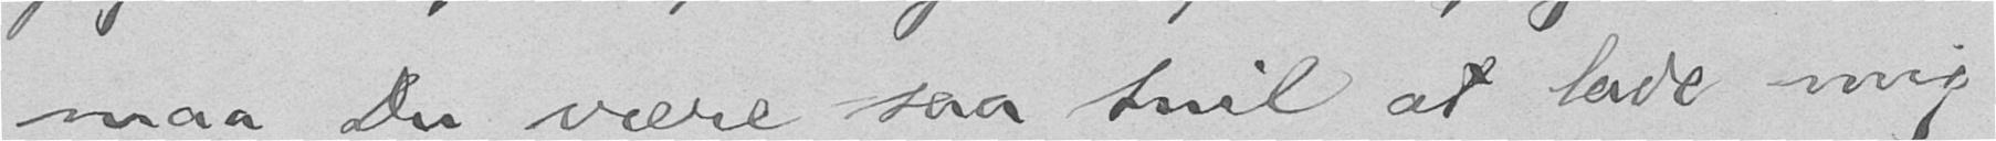maa Du være saa snil at lade mig
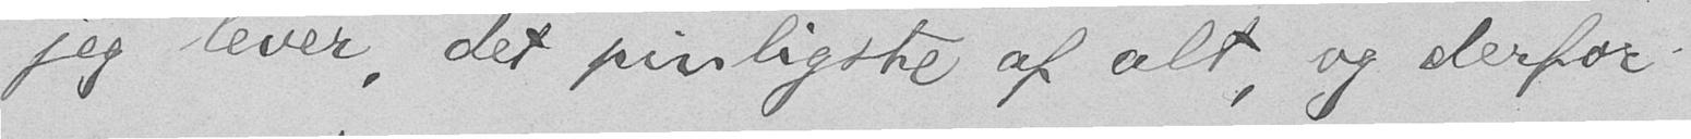jeg lever, det pinligste af alt, og derfor
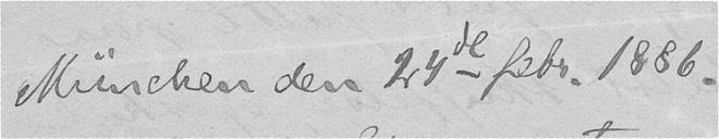München den 24de febr. 1886.
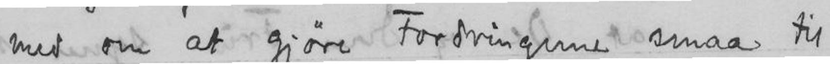med om at gjøre Fordringerne smaa til
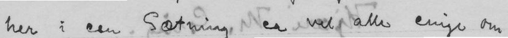her i den Sætning er vel alle enige om
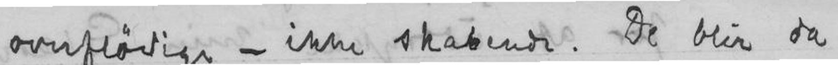overflødige - ikke skabende. De blir da
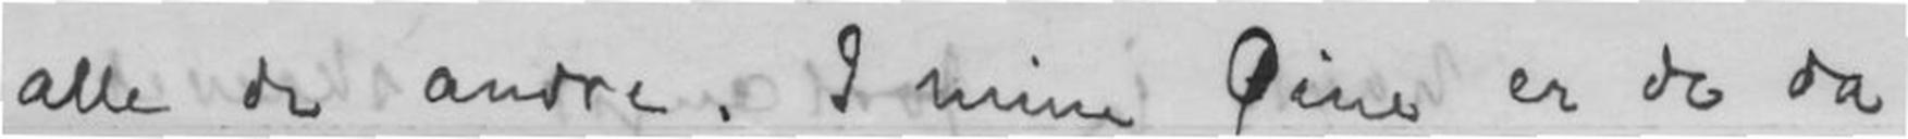alle de andre. I mine Øine er de da
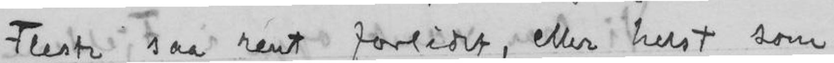Fleste saa rent forlidt, eller helst som
